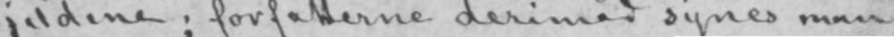fjeldene; forfatterne derimot synes man
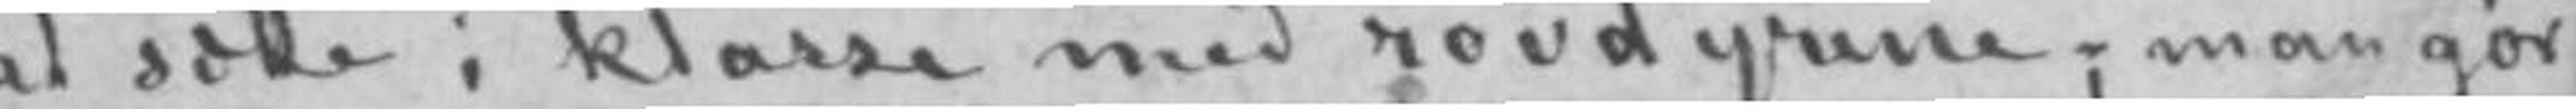at sætte i klasse med rovdyrene; man gør
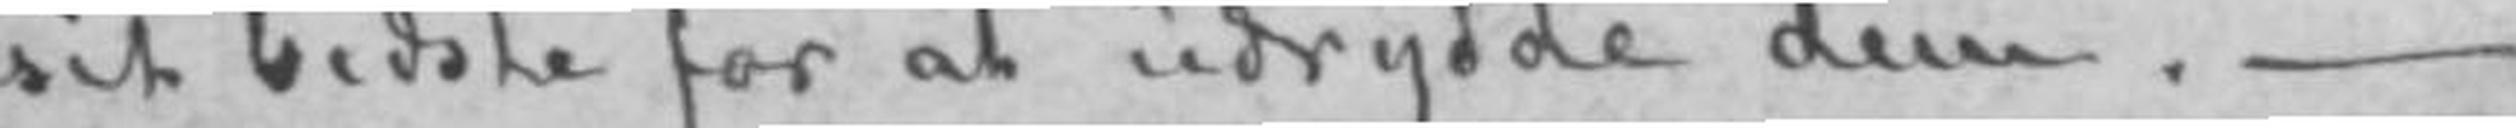sit bedste for at udrydde dem.-
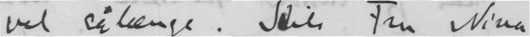vel sålænge. Hils Fru Nina
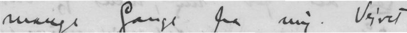mange Gange fra mig. Vejret
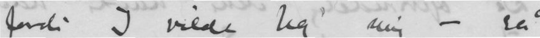fordi I vilde ha mig - så
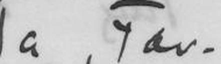Ja, Far-
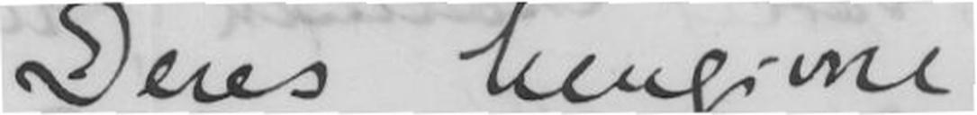Deres hengivne
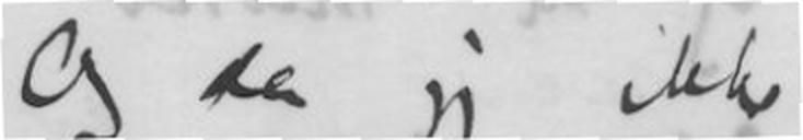Og da jeg ikke
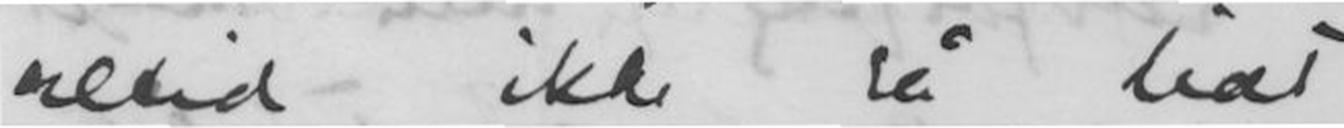altid ikke så lidt.
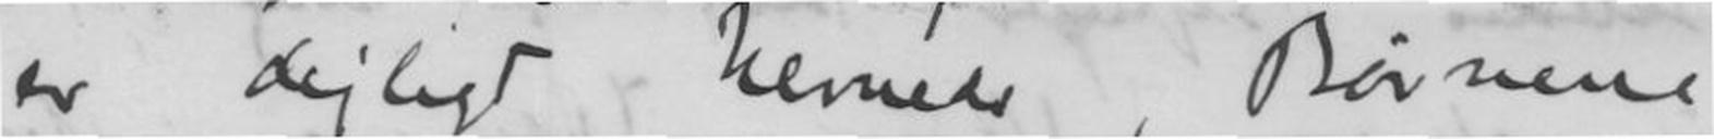er dejligt hernede, Børnene
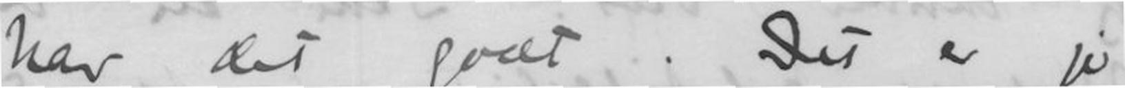har det godt. Det er jo
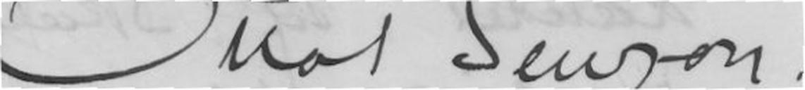Otto Benzon.
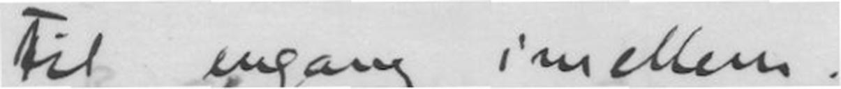til engang imellem.
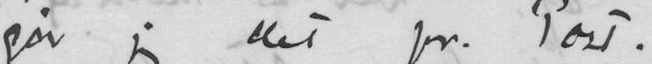gør jeg det pr. Post
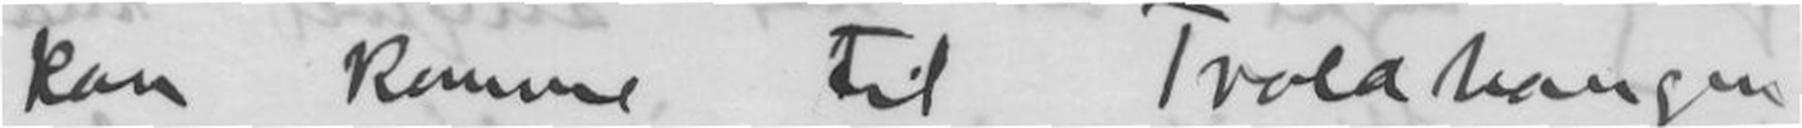kan komme til Troldhaugen -
